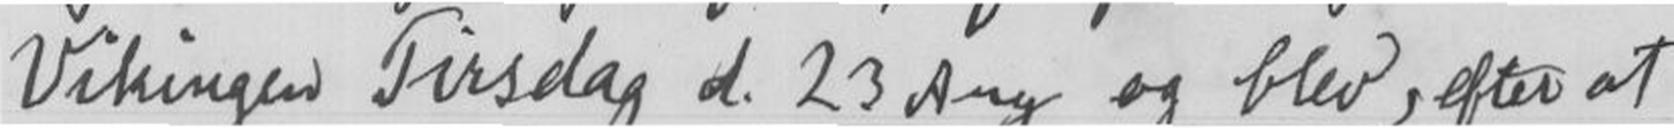Vikingen Tirsdag d. 23 Aug og blev, efter at
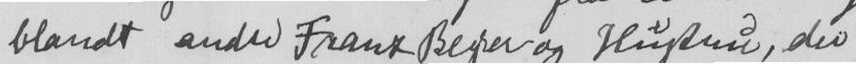blandt andre Franz Beyer og Hustru, der
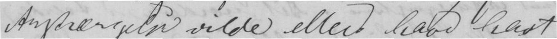Anstrængelse vilde eller have havt
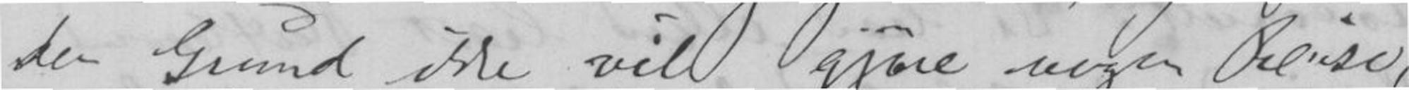den Grund ikke vil gjøre nogen Reise,
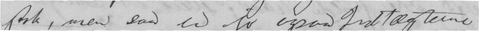stok, men saa er jo ogsaa Intægterne
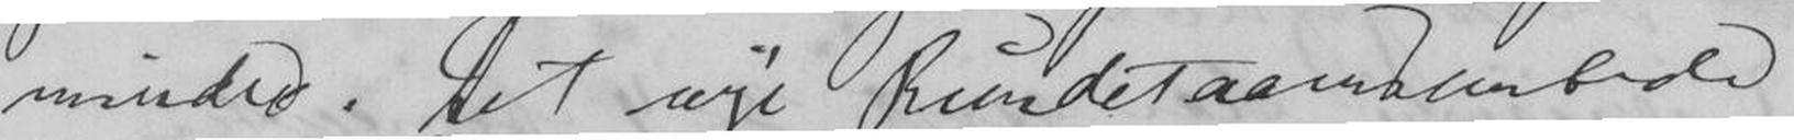mindre. Det nye Rundetaarnsembede
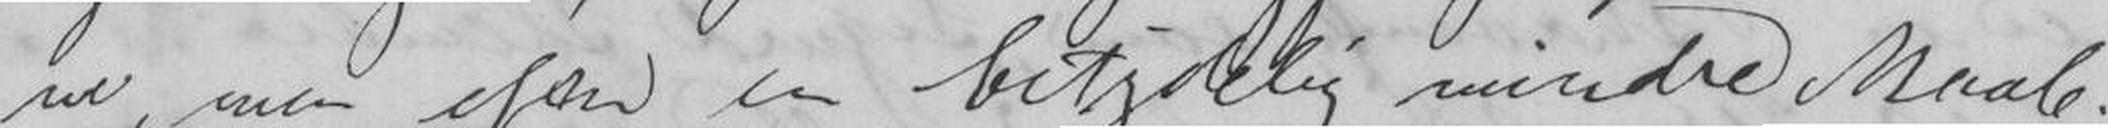ne, men efter en betydelig mindre Maale-
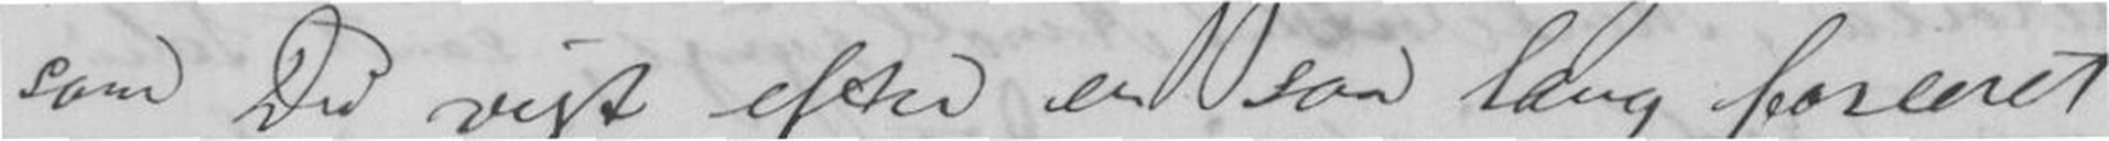som Du vist efter en saa lang forceret
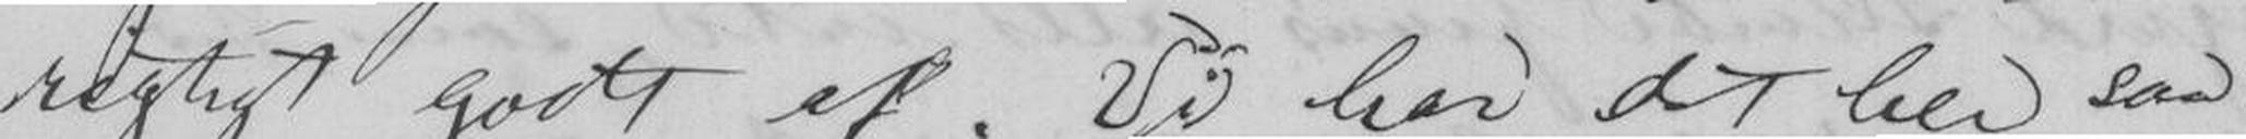rigtigt godt af. Vi har det her som
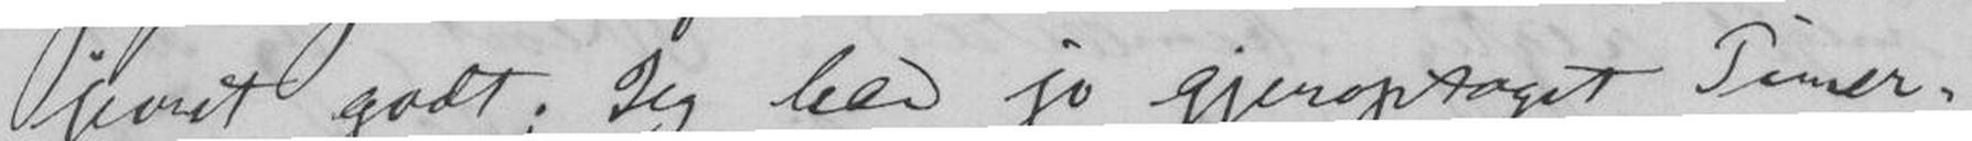jevnt godt; Jeg har jo gjenoptaget Timer-
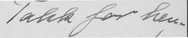Takk for hen-
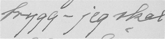trygg- jeg skal
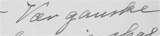Vær ganske
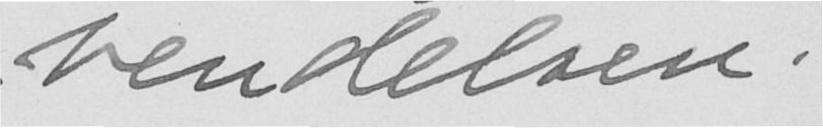vendelsen.
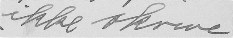ikke skrive
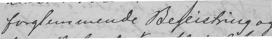forglemmende Begeistring og
